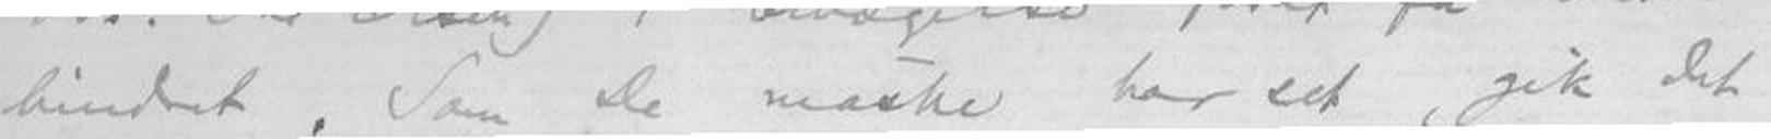hindret. Som De måske har set, gik det
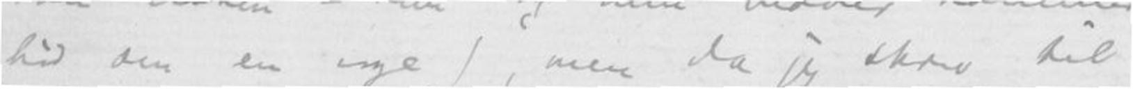hid om en uge, men da jeg skrev til
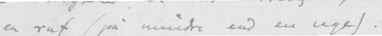en ruf (på mindre end en uge)
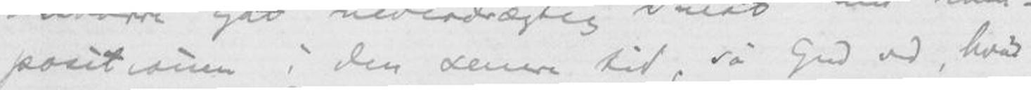positionen i den senere tid, så Gud ved, hvad
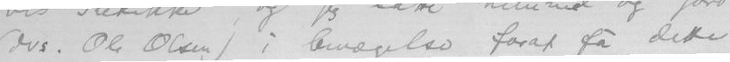(dvs. Ole Olsen) i bevægelse forat få dette
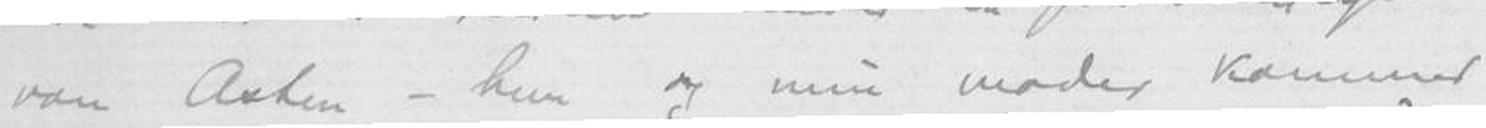von Asten - hun og min moder kommer
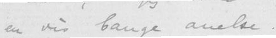en vis bange anelse.
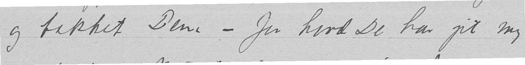og takket Dem – for hvad De har git mig
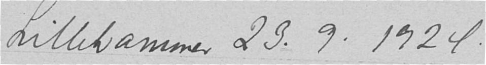Lillehammer 23.9.1924
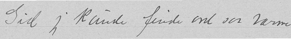Gid jeg kunde finde ord saa varme
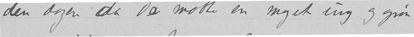den dagen da De møtte en meget ung og grøn
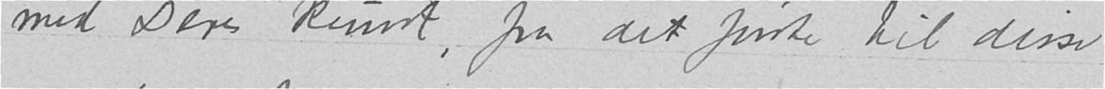med Deres kunst, fra det første til disse
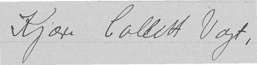Kjære Collett Vogt,
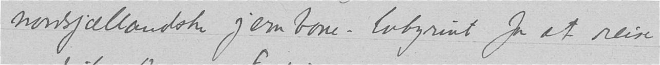nordsjællandske jernbane-labyrint for at reise
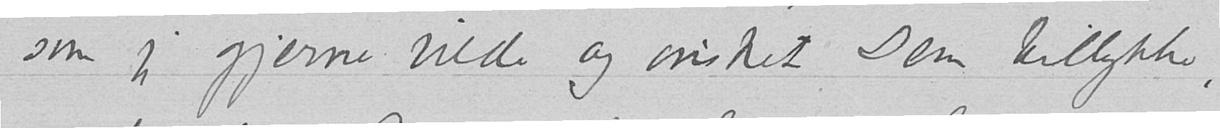som jeg gjerne vilde og ønsket Dem tillykke,
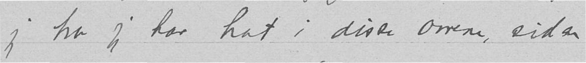jeg tror jeg har hat i disse aarene, siden
